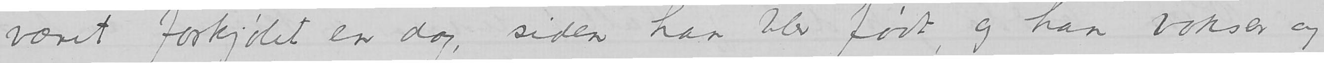været forkjølet en dag, siden han blev født, og han vokser og
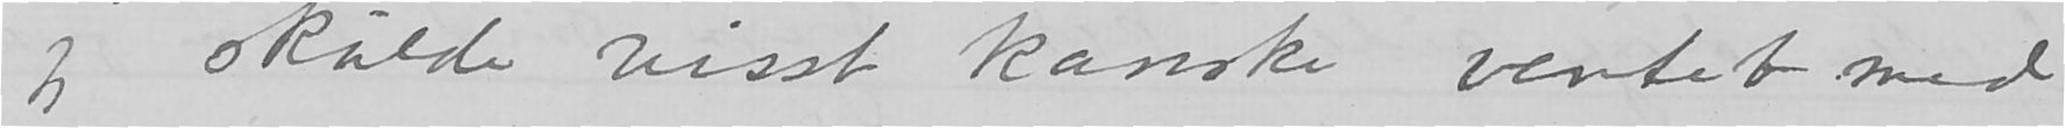jeg skulde visst kanske ventet med
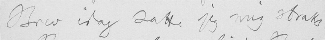Brev idag satte jeg mig straks
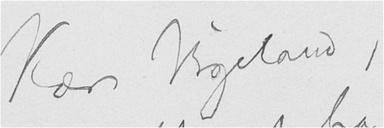Kære Vigeland,
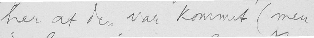her at den var kommet (men
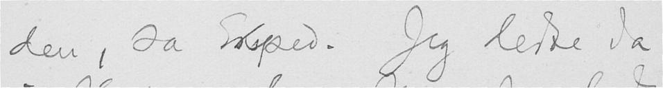den, sa Eksped. Jeg ledte da
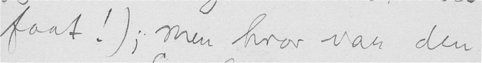faat!); men hvor var den
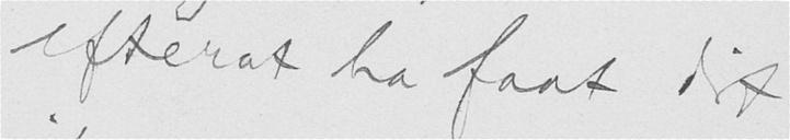efterat ha faat dit
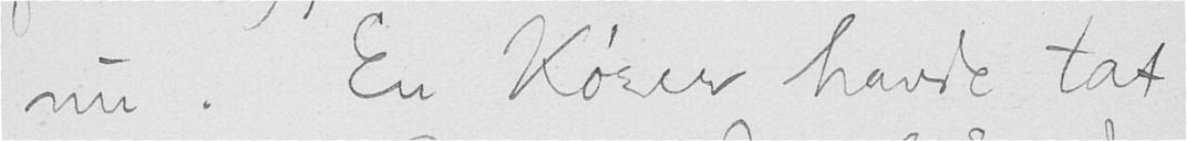nu. En Kører havde tat
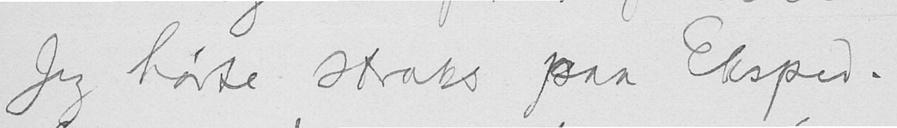Jeg hørte straks paa Eksped.
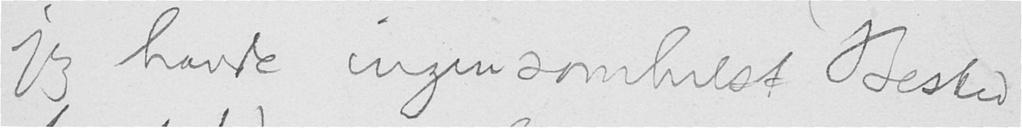jeg havde ingensomhelst Besked
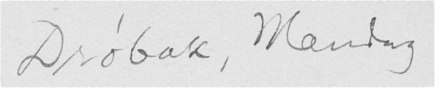Drøbak, Mandag
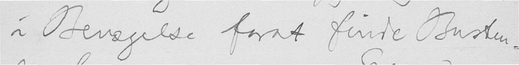i Bevægelse forat finde Busten.
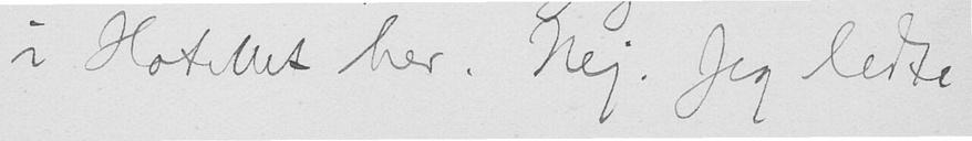i Hotellet her. Nej. Jeg ledte
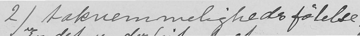2) taknemmelighedsfølelse.
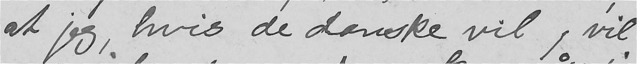at jeg, hvis de danske vil, vil
